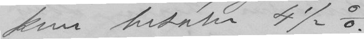kun betaler 4 1/2 %.
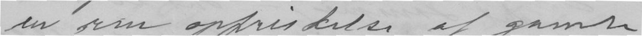en ren opfriskelse af gamle
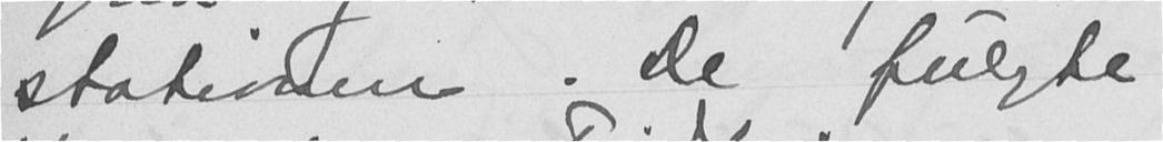stationen. De fulgte
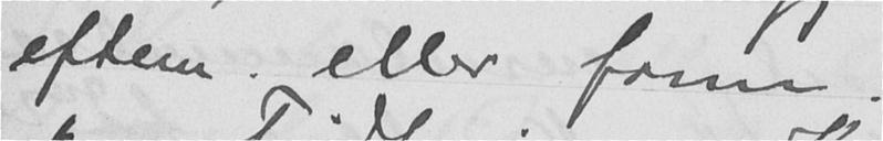efterm. eller form.
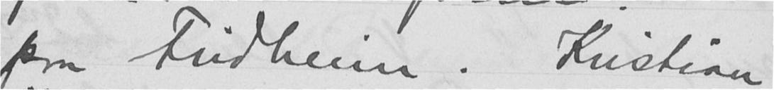paa Fridheim. Kristian
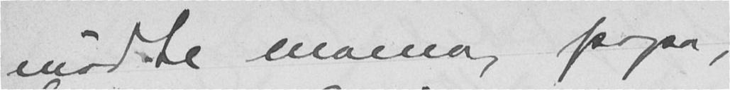mødte mama, papa,
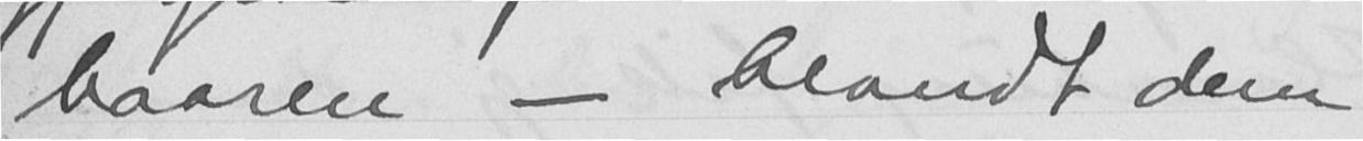baaren - blandt dem
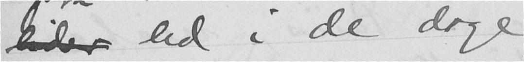lider led i de dage
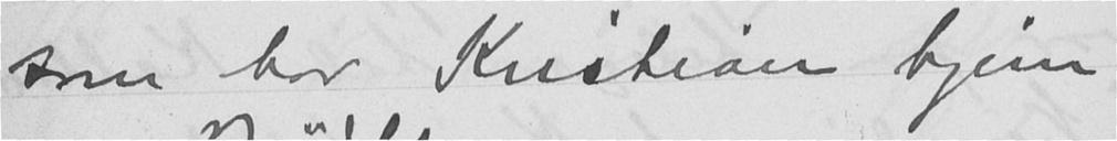som bar Kristian hjem
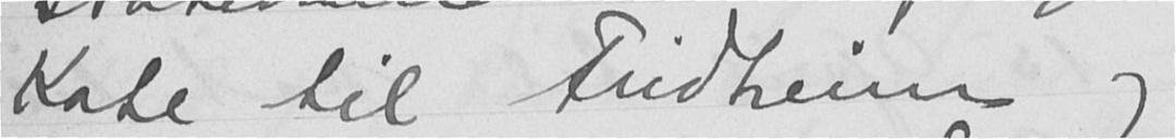Kate til Fridheim og
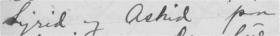Sigrid og Astrid paa
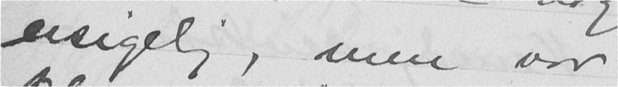usigelig, men var
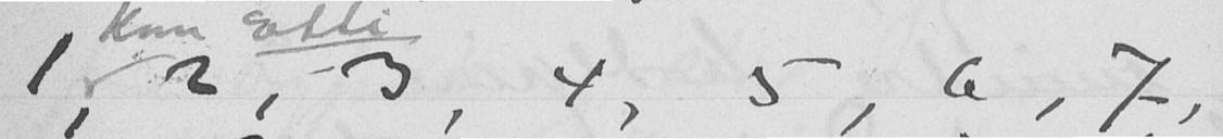1, 2, 3, 4, 5, 6, 7,
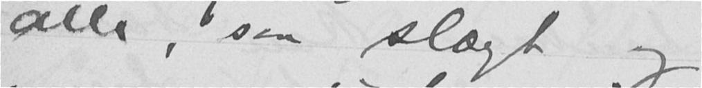alle, saa slægt og
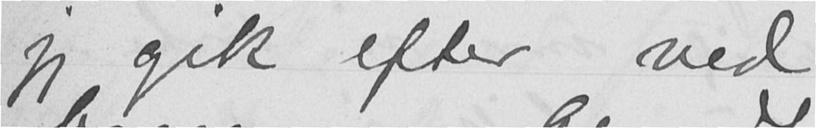jeg gik efter ved
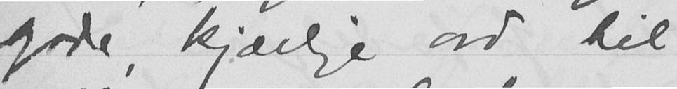gode, kjærlige ord til
